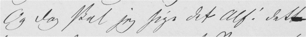Og dog skal jeg sige det Alf! Dette
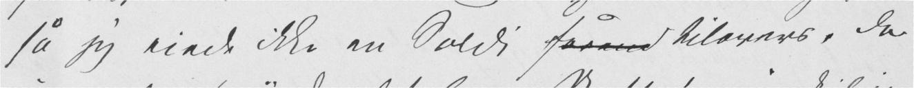så jeg eiede ikke en Soldi førend tilovers, der
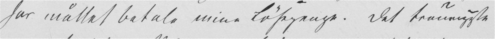har måttet betale mine Løsepenge. Det traurigste
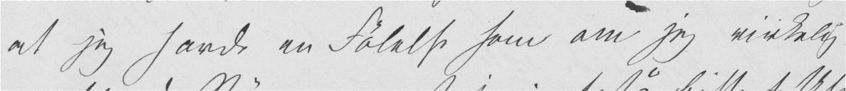at jeg havde en Følelse som om jeg virkelig
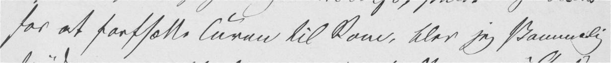for at fortsætte Turen til Rom, blev jeg skammelig
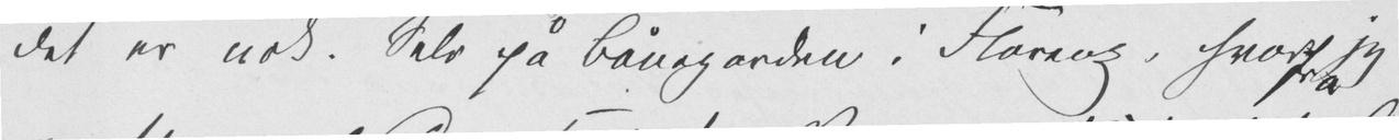det er nok. Selv på Banegarden i Florenz, hvor jeg
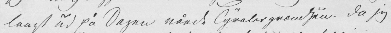langt ud på Dagen nåede Tyrolergrændsen. Da jeg
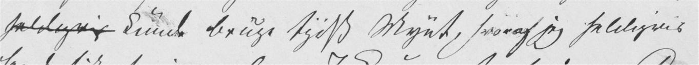heldigvis kunde bruge tydsk Mynt, hvoraf jeg heldigvis
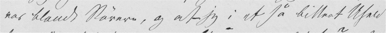var blandt Røvere, og at jeg i et så bittert Uheld
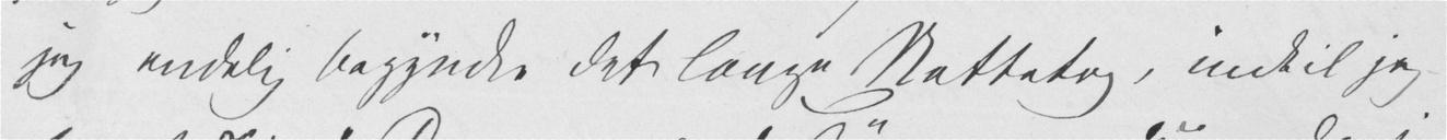jeg endelig begyndte det lange Nattetog, indtil jeg
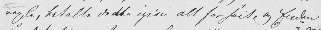vexle, betalte dette igjen alt for høit, og Enden
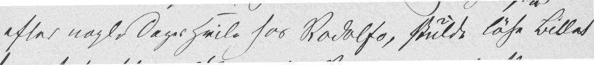efter nogle Dages Hvile hos Rodolfo, skulde løse Billet
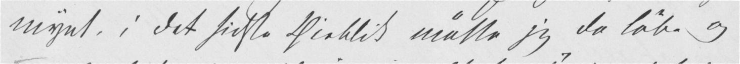mynt, i det sidste Øieblik måtte jeg da løbe og
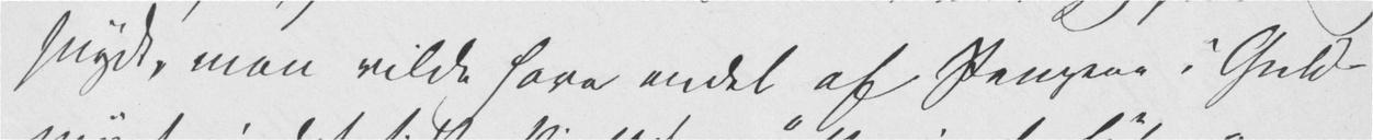snydt, men vilde have endel af Pengene i Guld-
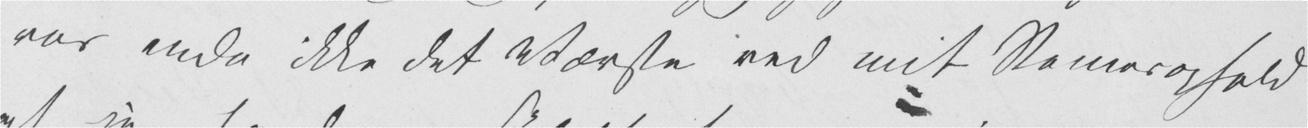var enda ikke det Værste ved mit Romerophold
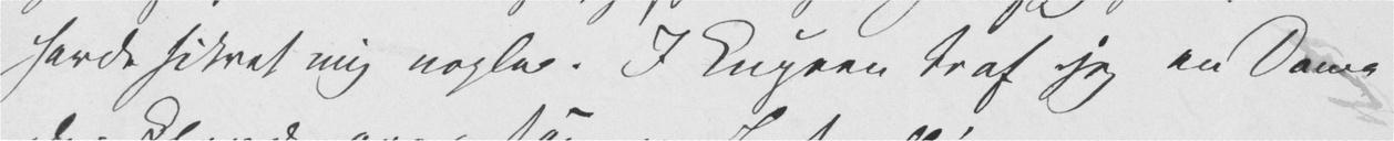havde sikret mig nogle. I Kupeen traf jeg en Dame
